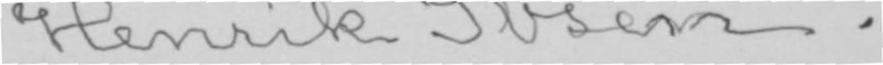Henrik Ibsen.
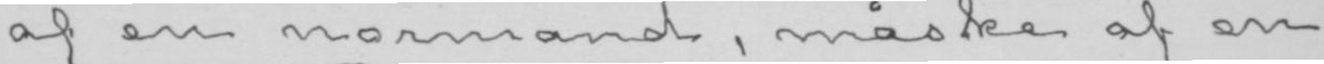af en normand, måske af en
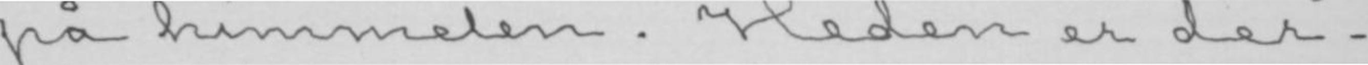på himmelen. Heden er der-
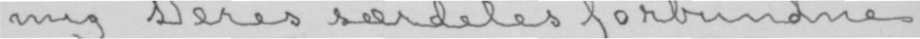migDeres særdeles forbundne
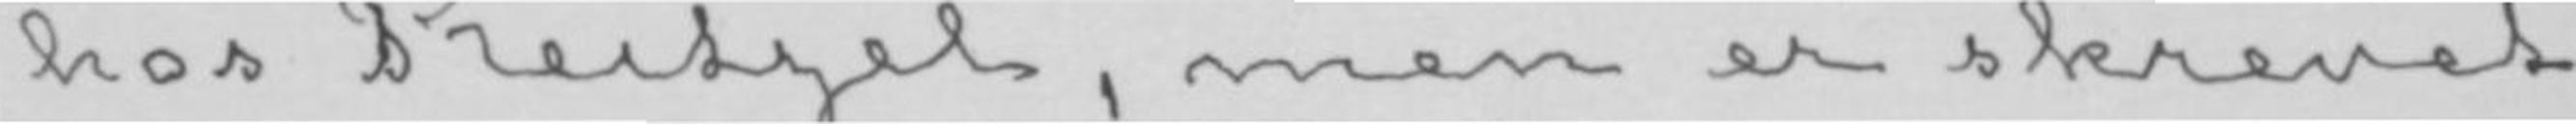hos Reitzel, men er skrevet
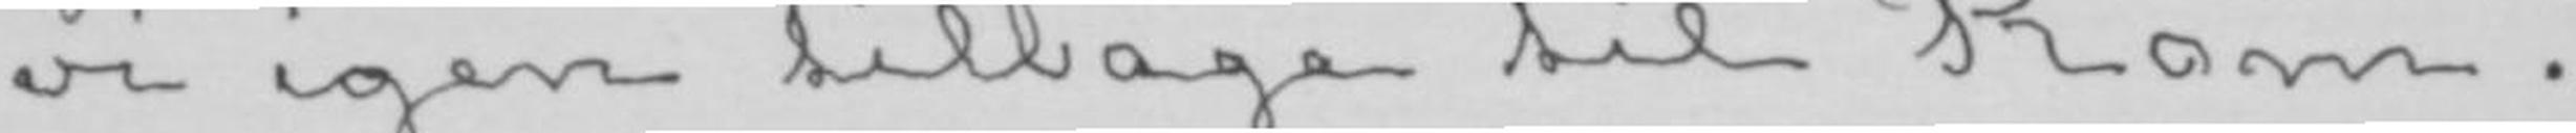vi igen tilbage til Rom.
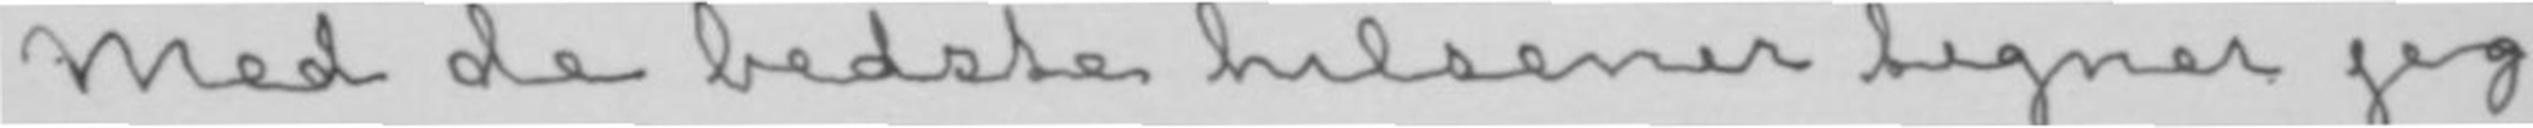Med de bedste hilsener tegner jeg
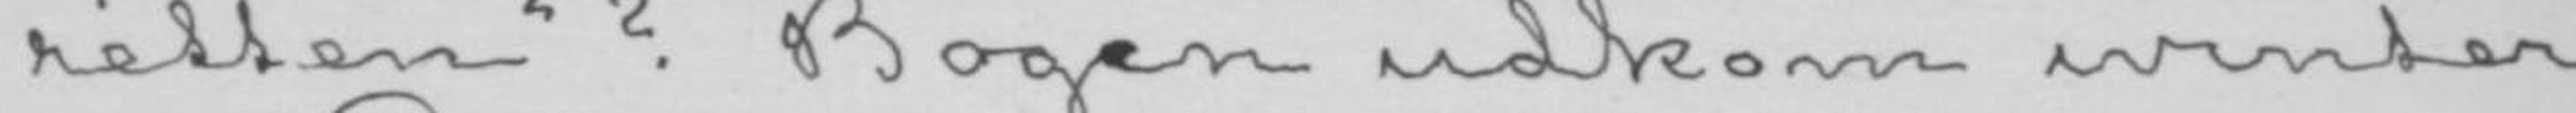retten»? Bogen udkom ivinter
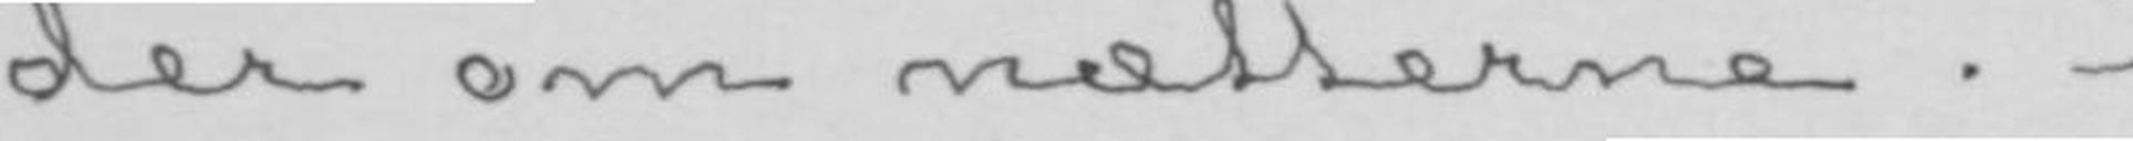der om nætterne.-
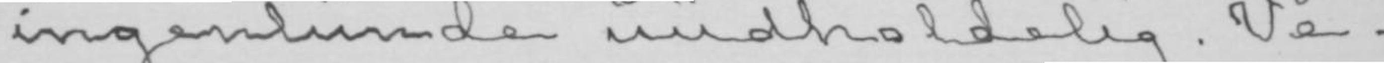ingenlunde uudholdelig. Ve-
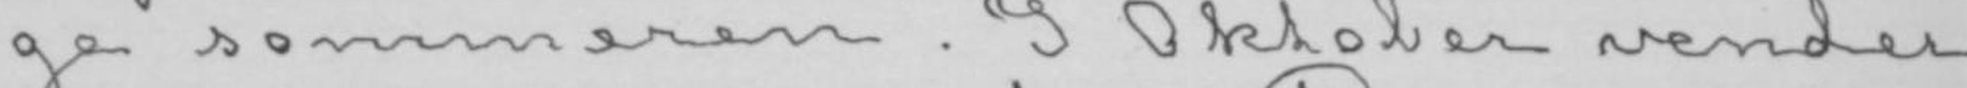ge sommeren. I Oktober vender
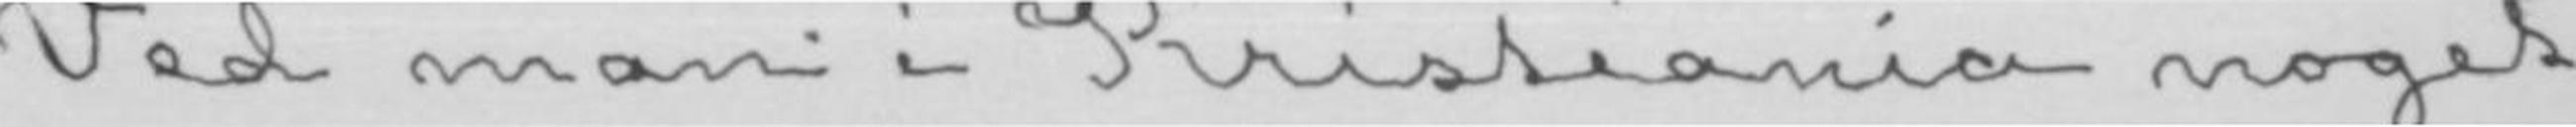Ved man i Kristiania noget
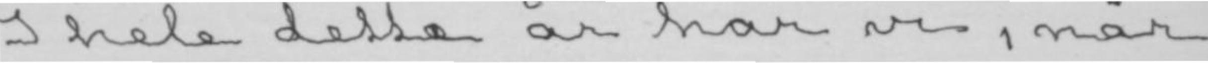I hele dette år har vi, når
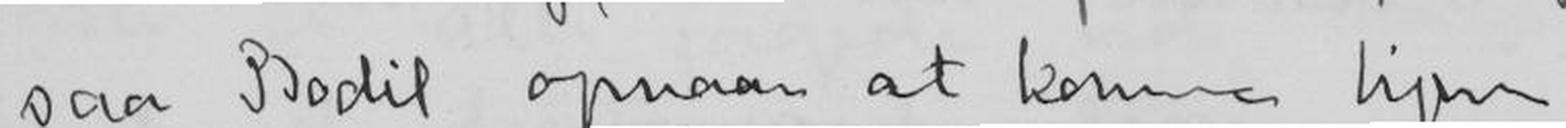saa Bodil opnaar at komme hjem
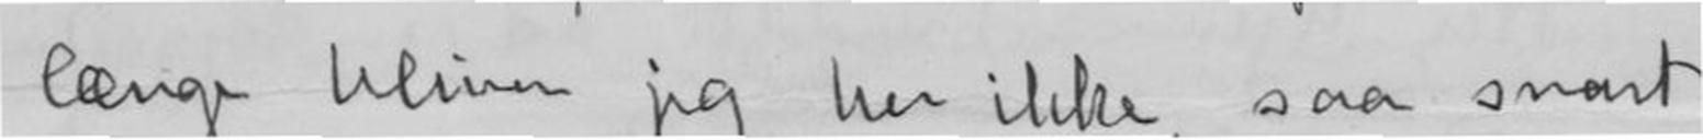længe bliver jeg her ikke, saa snart
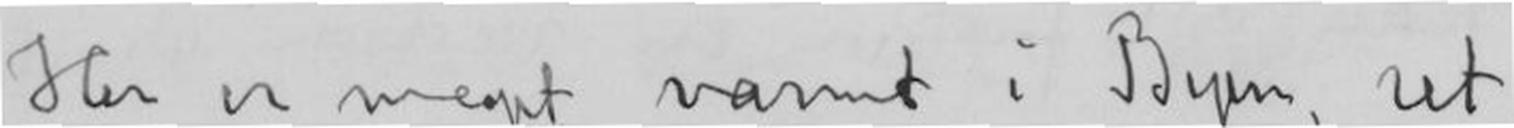Her er meget varmt i Byen, ret
